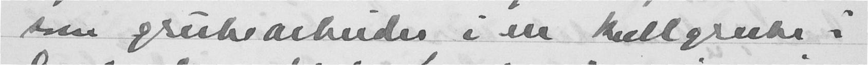som gruvearbeider i en kullgrube i
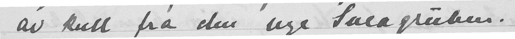av kull fra den nye Sveagruben.
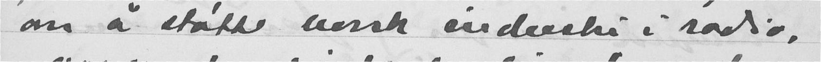om å skaffe norsk industri i radio,
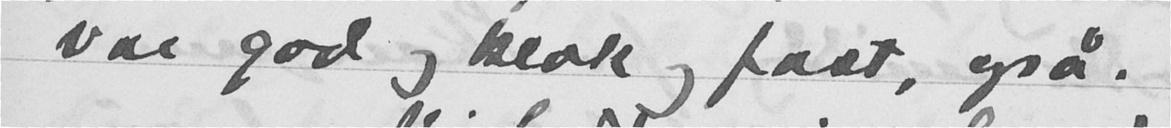var god og klok og fast, også.
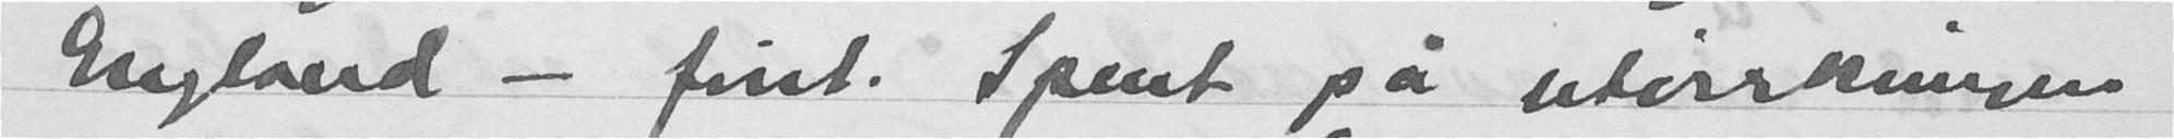England - fint. Spent på utvinning
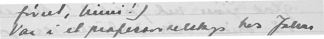Var i et professorselskap hos Johan
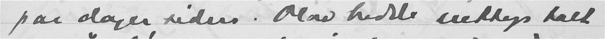par dager siden. Olav hadde nettop talt
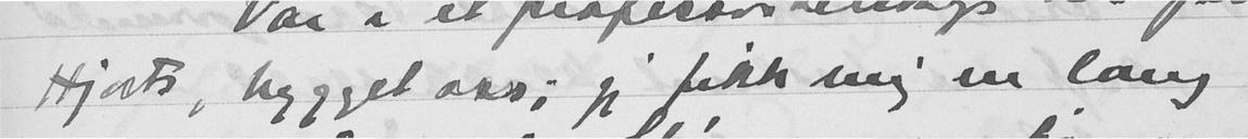Hjorth, hygget oss; jeg fikk mig en lang
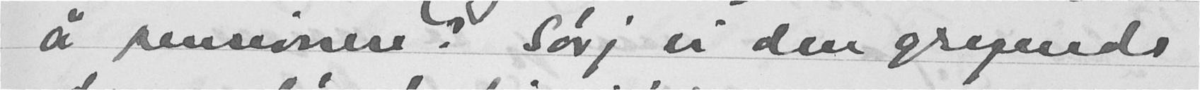å pensionere? Sørj ei den gryende
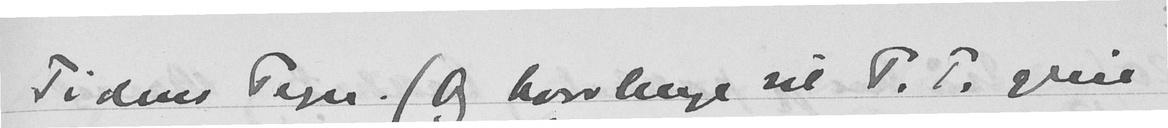Tidens Tegn. (Og hvor lenge til T. T. greier
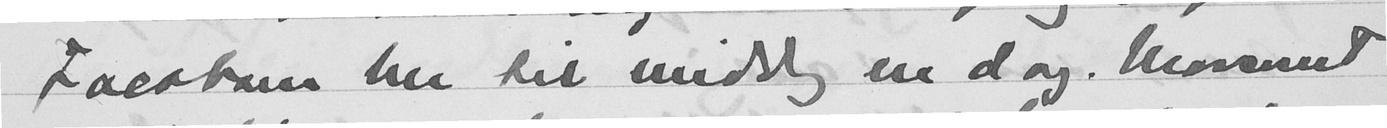Faerhavn her til middag en dag. Morsomt
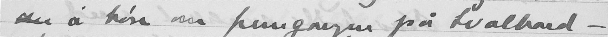å høre om fremgangen på Svalbard -
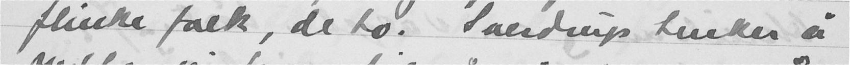flinke folk, de to. Sverdrup tenker å
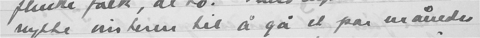nytte vinteren til å gå et par måneder
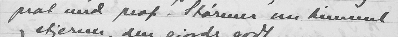prat med prof: Størmer om himmel
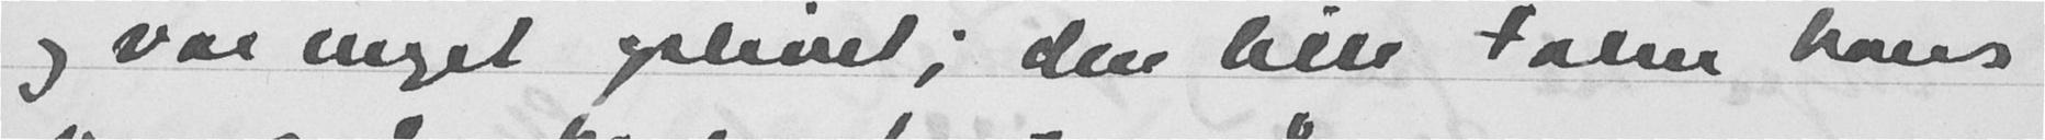og var meget oplivet; den lille talen hans
